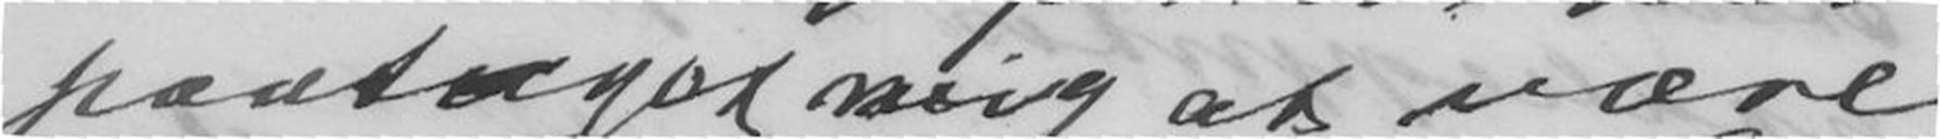paataget mig at være
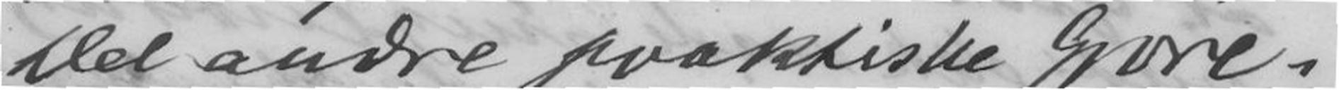Del andre praktiske Gjøre-
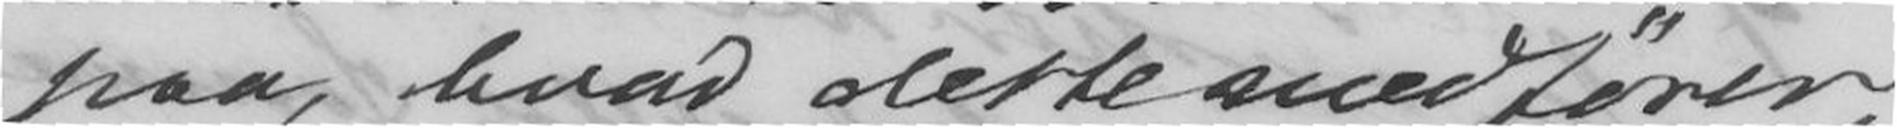paa, hvad dette medfører,
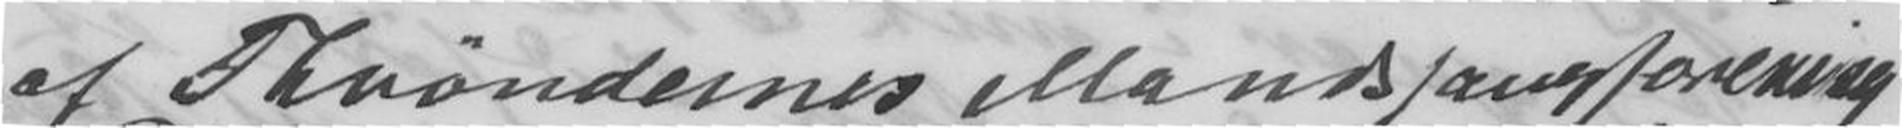af Thrøndernes Mandssangforening.
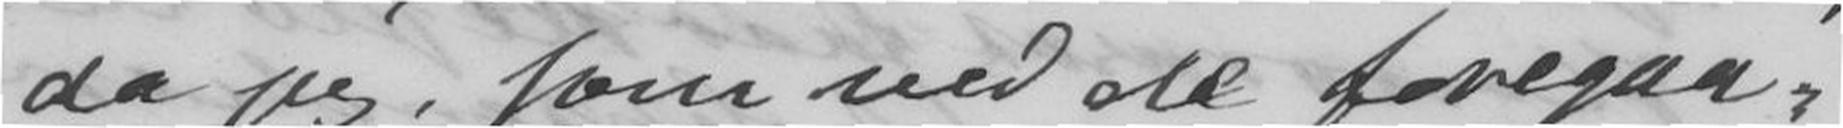da jeg, som ved all foregaa-
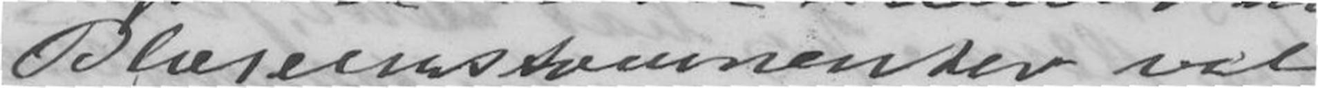Blæseinstrumenter vil
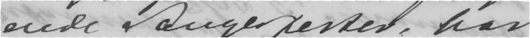ende Sangerfesten, har
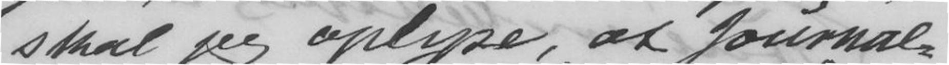skal jeg oplyse, at Journal-
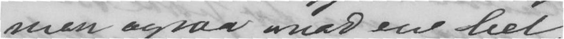men ogsaa mod en hel
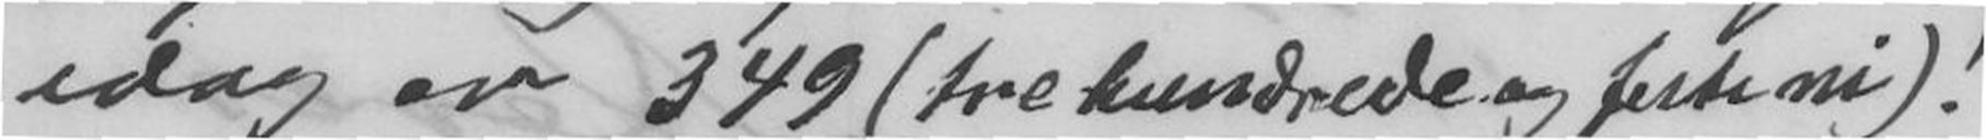idag er 349 (tre hundrede og firti ni)!
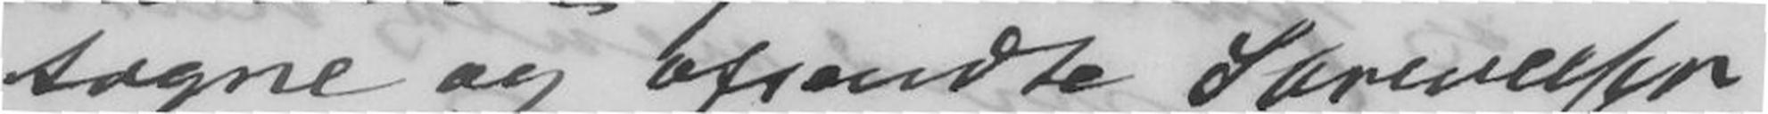tagne og afsendte Skrivelser
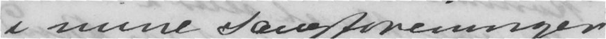i mine Sangforeninger
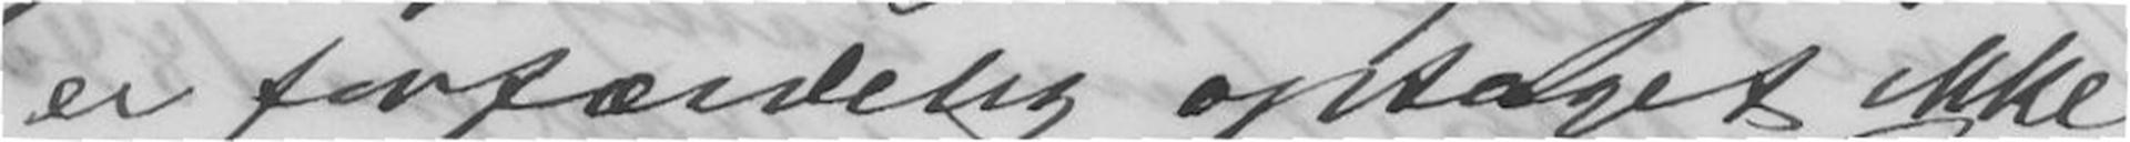er forfærdelig optaget ikke
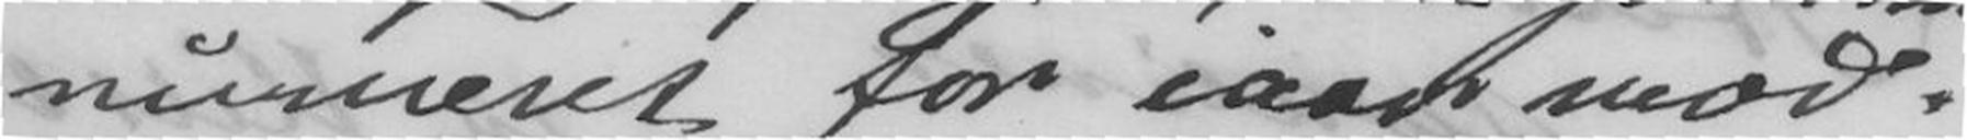numeret for iaar mod-
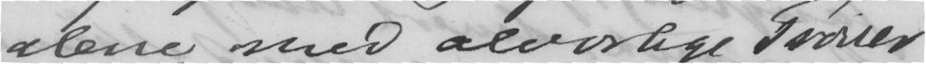alene med alvorlige Teorier
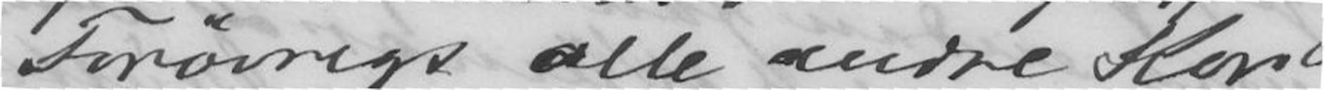Forøvrigt alle andre Kor
